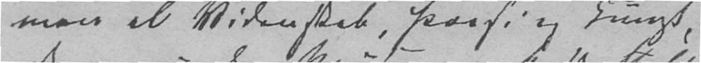men al Videnskab, Poesi og Kunst,
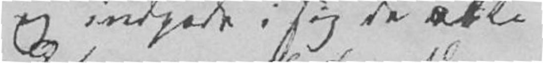og indpode i sig de ædle
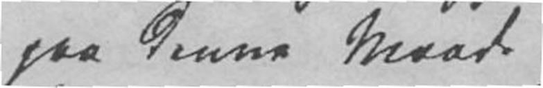paa denne Maade
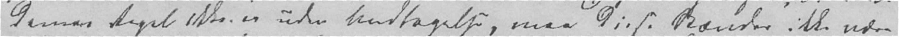denne Regel ikke er uden Undtagelse, maa disse Stænder ikke være
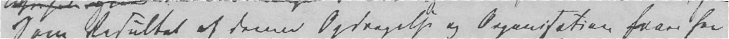Som Resultat af denne Opdragelse og Organisation faaer hun
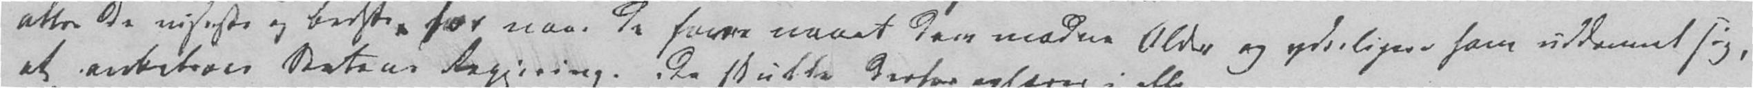atter de viseste og bedste, for naar de have naaet den modne Alder og yderligere have uddannet sig,
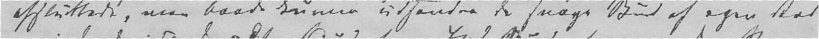aflukkede, men baade kunne udsondre de svage Skud af egen Stat
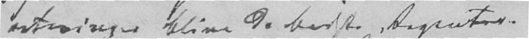retninger blive de bedste Regenter.
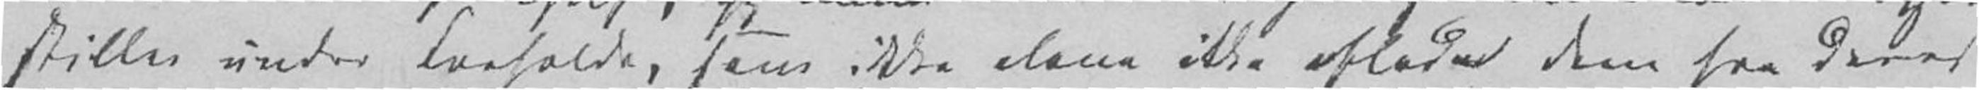stilles under Forholde, som ikke alene ikke aflader dem fra deres
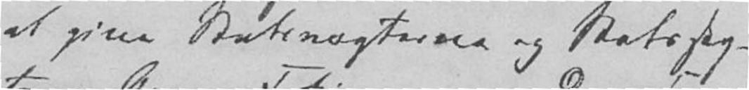at give Statsvogterne og Statssty-
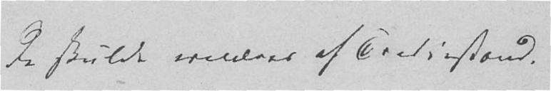de skulde ernæres af Trediestand.
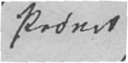Prøver
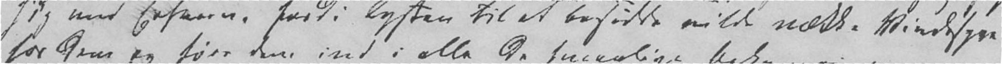sig med Erhverv, fordi Lysten til at besidde vilde vække Vindesyge
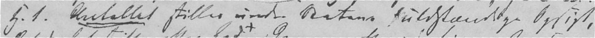H. t. Antallet stilles under Statens fuldstændige Opsigt,
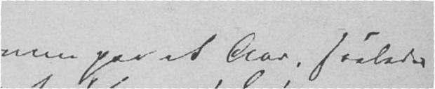men paa et Aar, saaledes
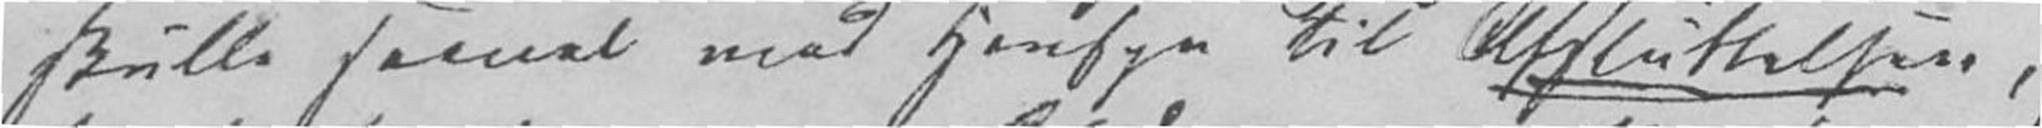skulle saavel med Hensyn til Afsluttelsen,
